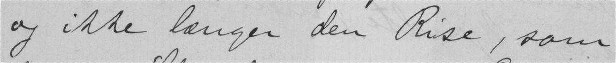og ikke længer den Rise, som
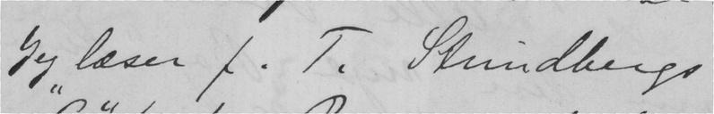Jeg læser f. T. Strndbergs
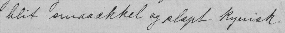blit smaaækkel og slapt kynisk.
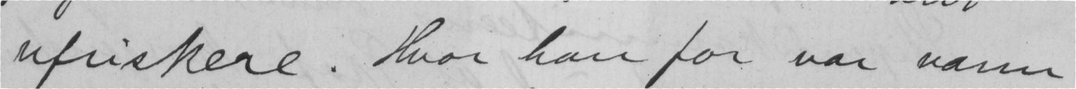ufriskere. Hvor han før var harm
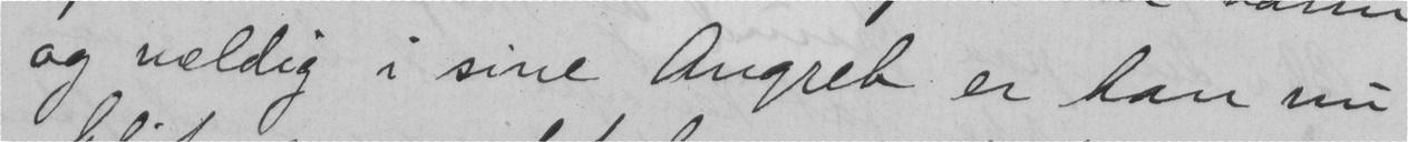og veldig i sine Angreb er han nu
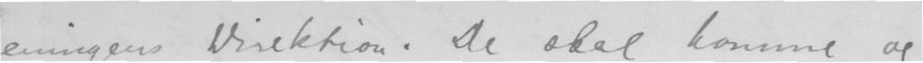eningens Direktion. De skal komme og
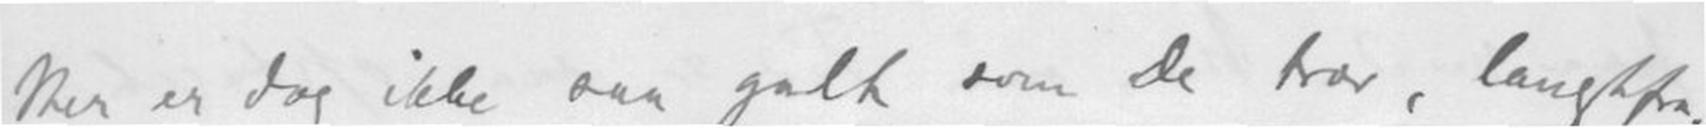Her er dog ikke saa galt som de tror, langtfra
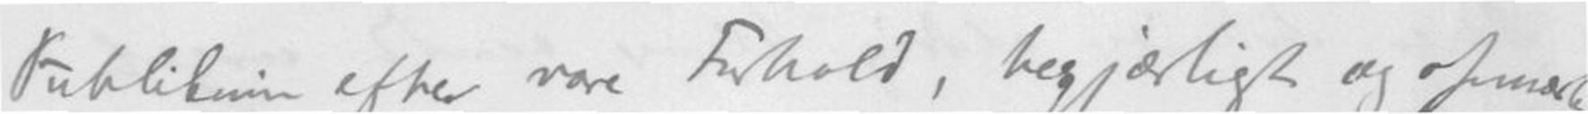Publikum efter vore Forhold, besjærligt og opmærk-
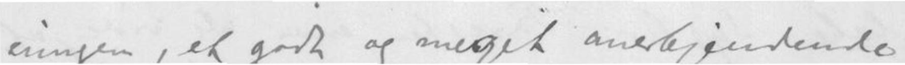eningen, et godt og meget andkjendende
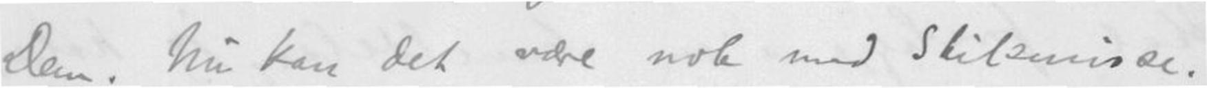Dem. Nu kan det være nok med Skilsmisse.
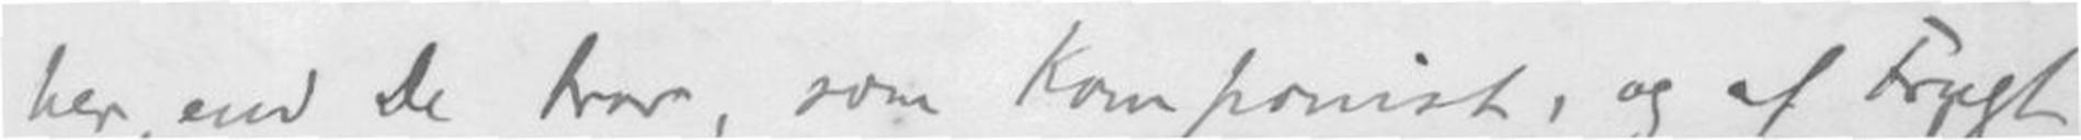her, end de tror, som komponist, og af Frygt
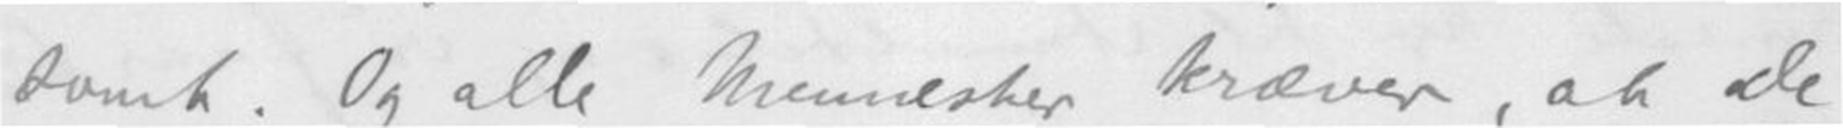somt. Og alle Mennesker kræver, at de
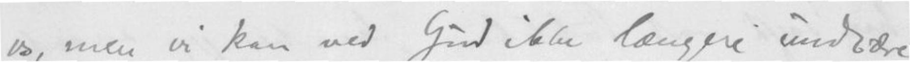os, men vi kan ved Gud ikke længere undvære
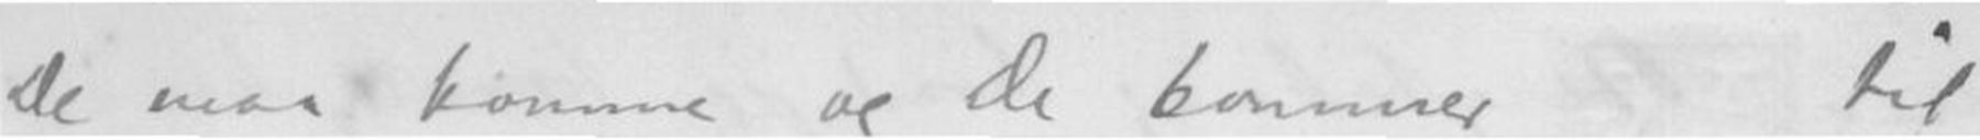de skal komme og de kommer til
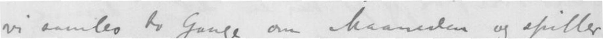vi samles to Ganger om Maaneden og spiller
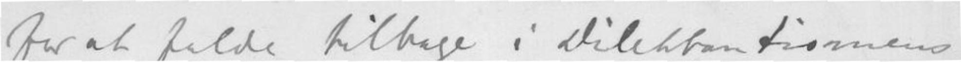for at falde tilbage i Dillettantismens
